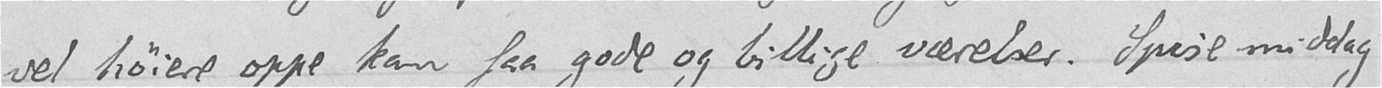vel høiere oppe kan faa gode og billige værelser. Spise middag
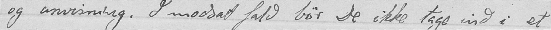og anvisning. I modsat fald bør De ikke tage ind i et
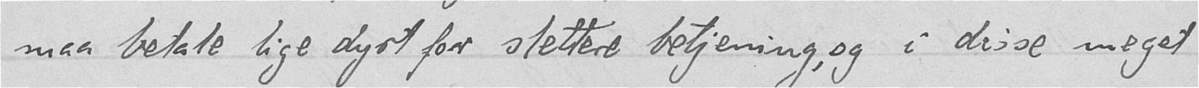maa betale lige dyrt for slettere betjening, og i disse meget
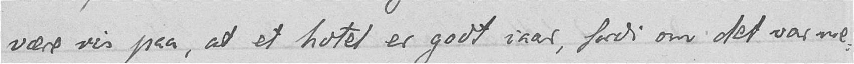være vis paa, at et hotel er godt iaar, fordi om det var me-
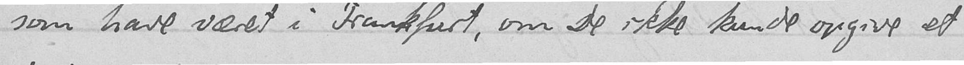som have været i Frankfurt, om De ikke kunde opgive et
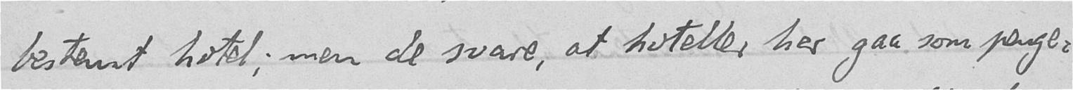bestemt hotel; men de svare, at hoteller her gaa som penge-
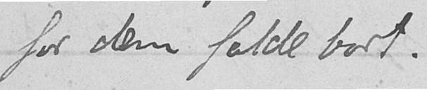for dem falde bort.
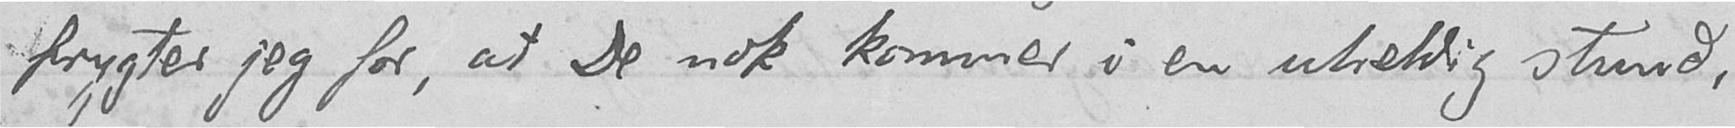frygter jeg for, at De nok kommer i en uheldig stund,
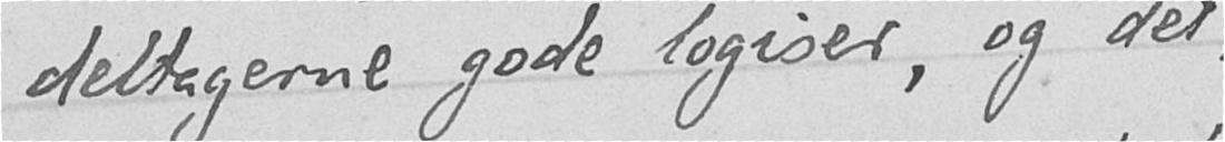deltagerne gode logiser, og det
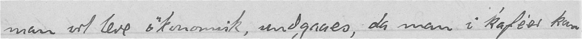man vil leve økonomisk, undgaaes, da man i kaféer kan
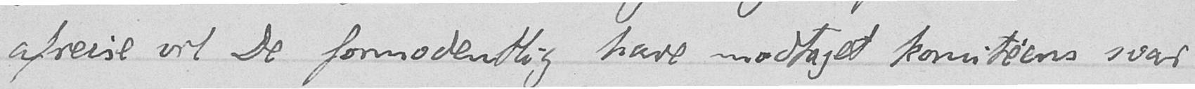afreise vil De formodentlig have modtaget komitéens svar
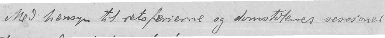Med hensyn til retsferierne og domstolernes sessioner
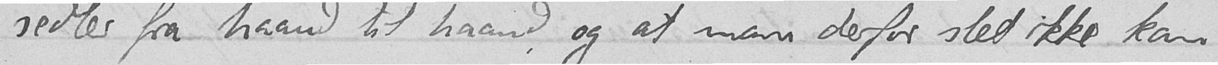sedler fra haand til haand, og at man derfor slet ikke kan
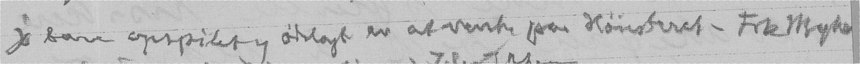jo bare opspilet og ødelagt av at vente paa Høiesteret. Frk Myhre
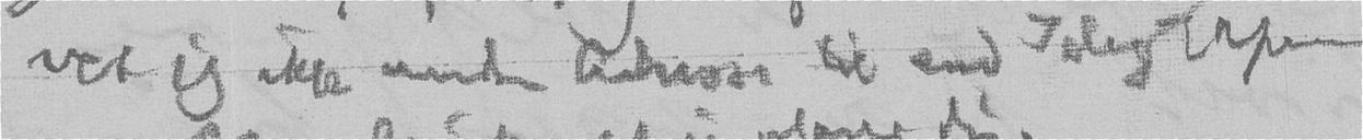vet jeg ikke anden Adresse til end Telegrafen
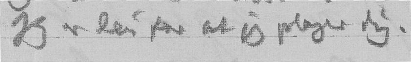Jeg er lei for at jeg plager dig.
